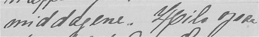middagene. Hils ogsaa
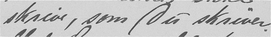skrive, som Du skriver.
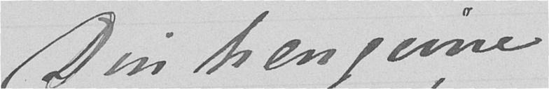Din hengivne
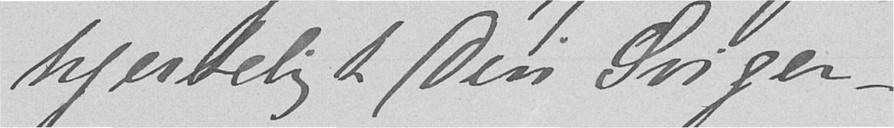hjerteligt Din Sviger-
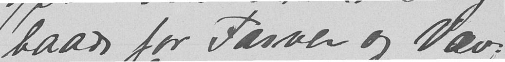baade for Farver og Væv;
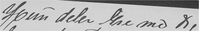Hun deler Sre med dig
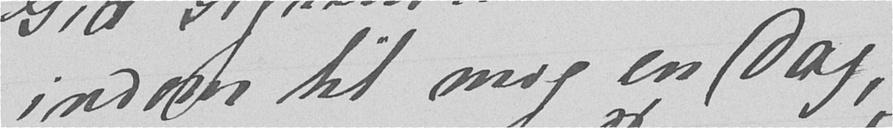indom til mig en Dag,
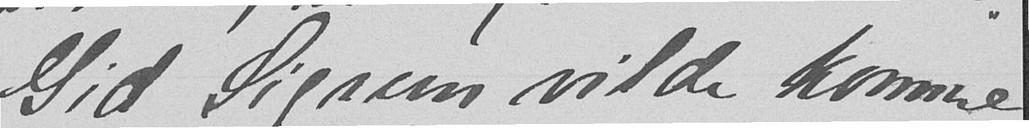Gid Sigrun vilde kommer
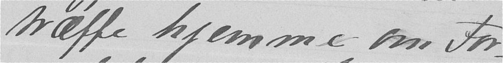træffe hjemme om For-
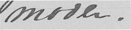moder.
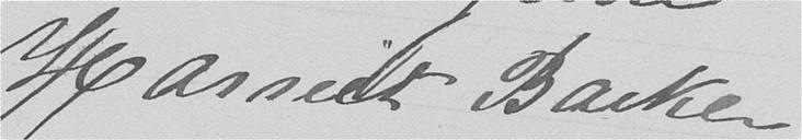Harriet Backer
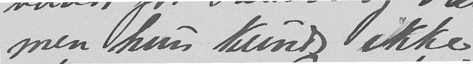men hun kunde ikke
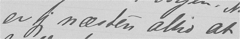er jeg næsten altid at
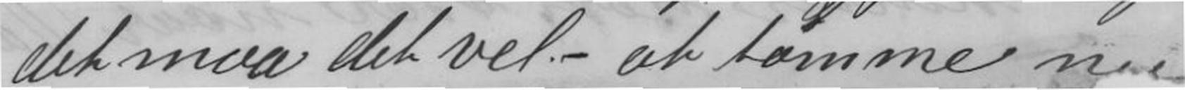det maa det vel - at tømme
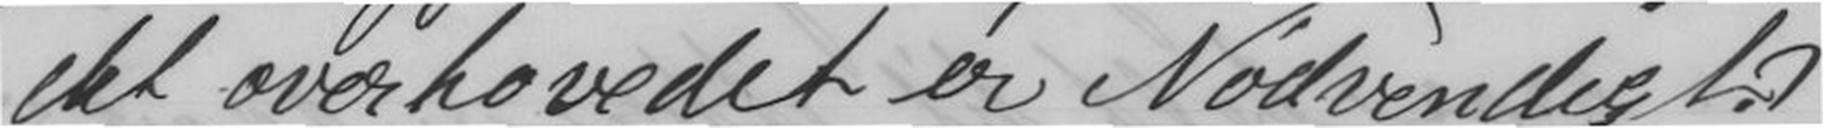det overhovedet er Nødvendigt.
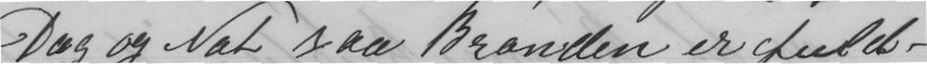Dag og Nat saa Brønden er fuld -
